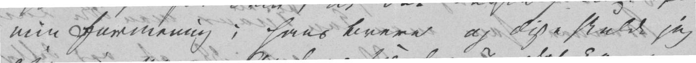min Formening i hans Breve og disse skulde jeg
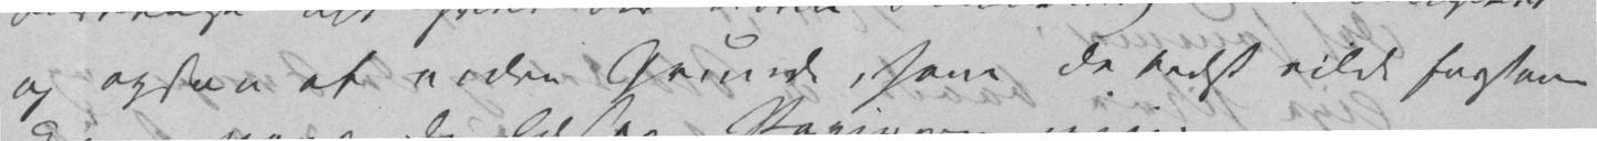og ogsaa af andre Grunde, som De bedst vilde forstaae
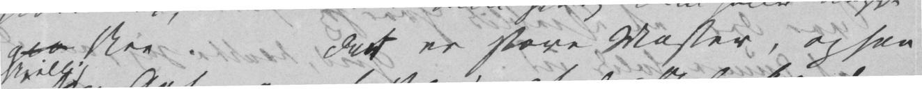gjø skee. Der er store Masser, og saa
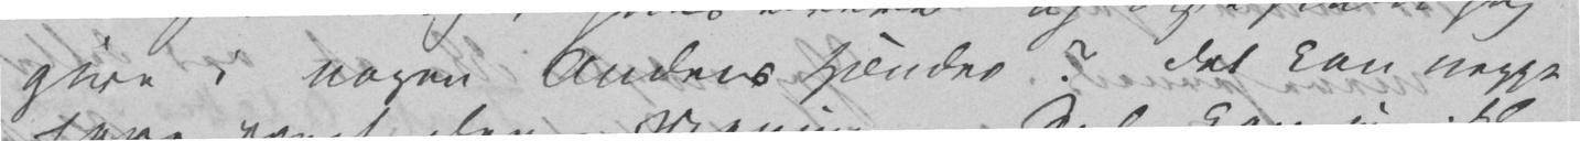give i nogen Andens Hænder? Det kan neppe
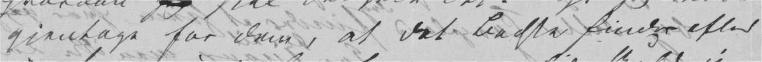gjentage for Dem, at det Bedste findes efter
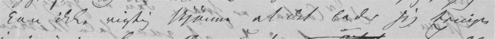kan ikke rigtig skjønne at det lader sig tvinge
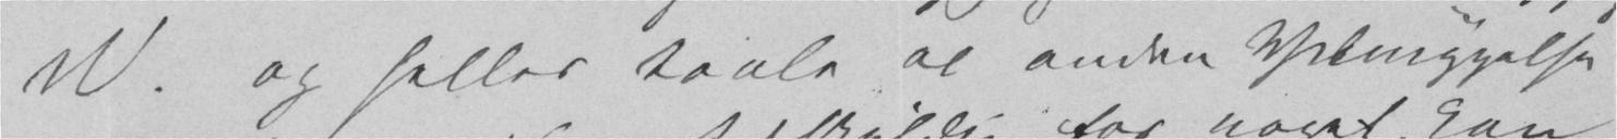W. og heller taale al anden Ydmygelse
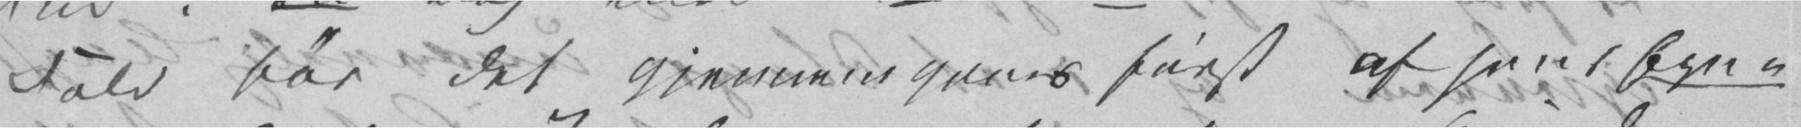Fald bør det gjennemgaaes først af hans Egne
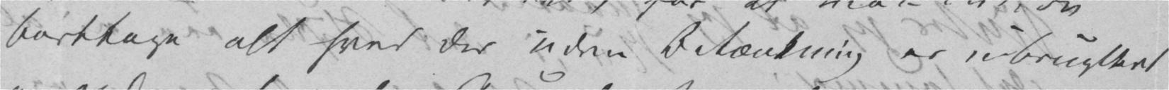borttage alt hvad der uden Betænkning er ubrugbart
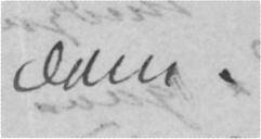dem.
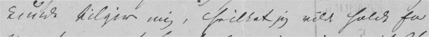kunde tilgive mig, hvilket jeg vilde holde for
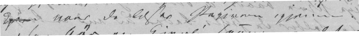kjere naar De læser Papirerne igjennem.
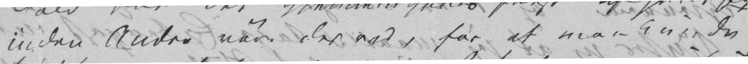inden Andre røre der ved, for at man kunde
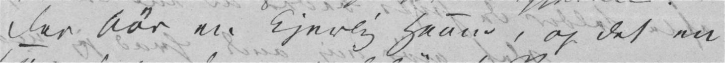Der bør en kjerlig Haand, og det en
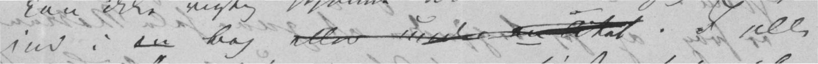ind i en Bog eller under en Titel. I alle
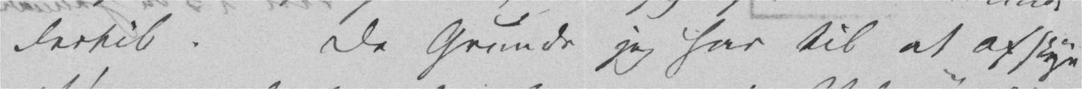dertil. De Grunde jeg har til at afskye
